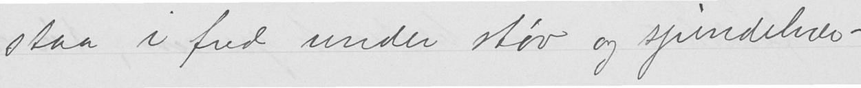staa i fred under støv og spindelvev -
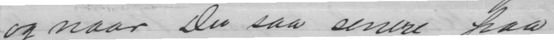og naar Du saa senere paa
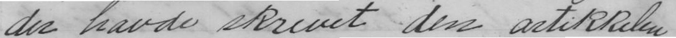der havde skrevet den artikkelen
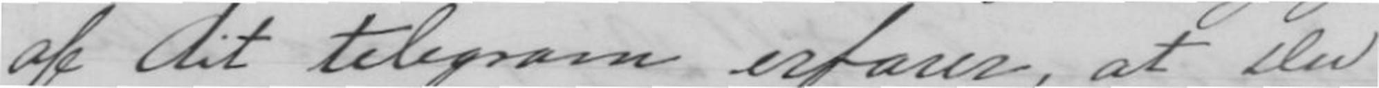af dit telegram erfarer, at Du
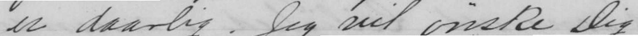er daarlig. Jeg vil ønske Dig,
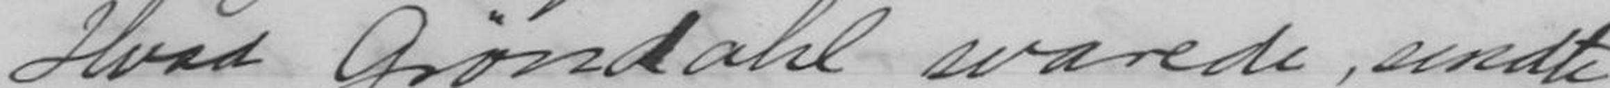Hvad Grøndahl svarede, sendte
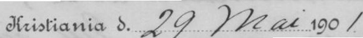Kristiania d. 29 Mai 1901.
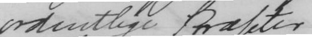ordentlige kræfter. -
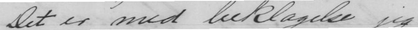Det er med beklagelse jeg
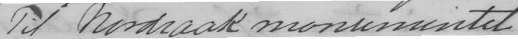Til Nordraak monumentet
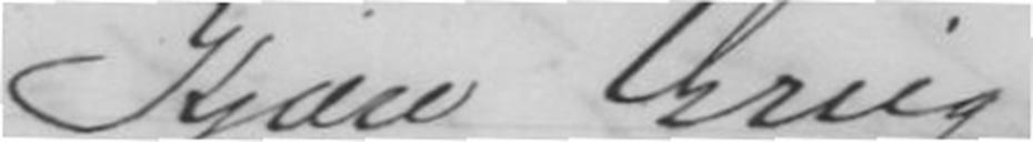Kjære Grieg!
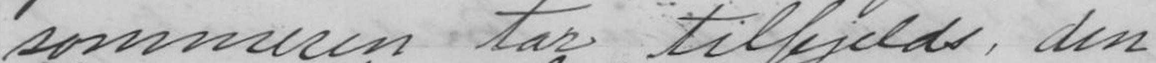sommeren tar tilfjelds, den
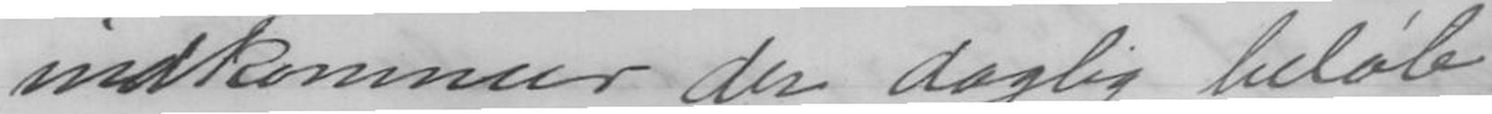indkommer der daglig beløb
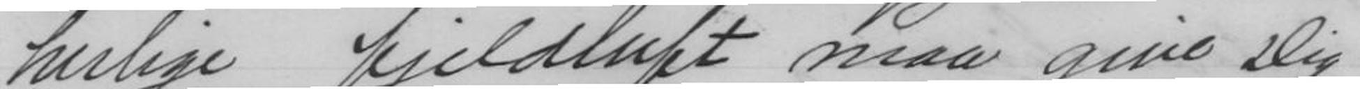herlige fjeldluft maa give Dig
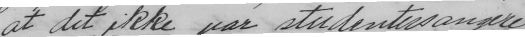at det ikke var studentersangere
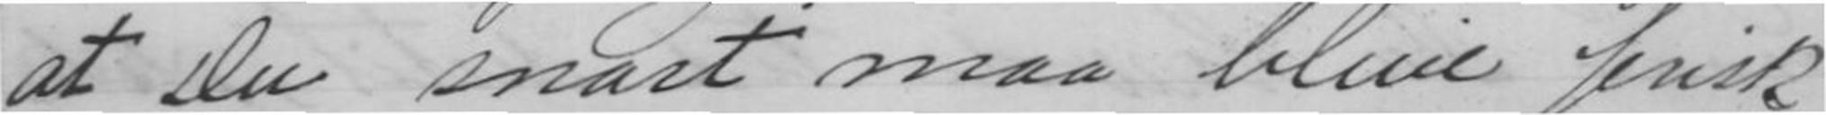at Du snart maa blive frisk
